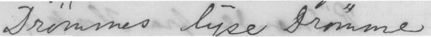Drømmes Lyse Drømme
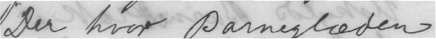Der hvor Barneglæden
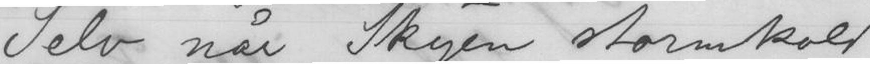Selv når Skyen stormkold
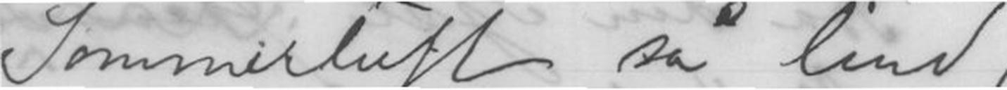Sommerluft så lind,
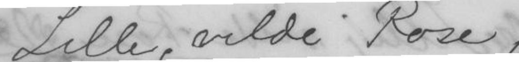Lille, vilde Rose,
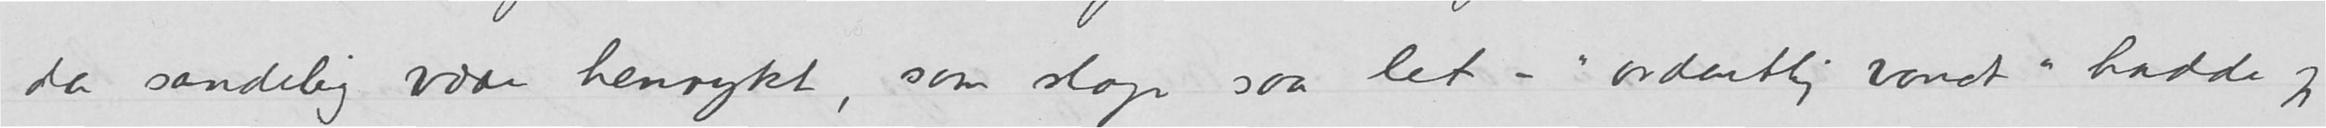da sandelig være henrykt, som slap saa let – «ordentlig vondt» hadde jeg
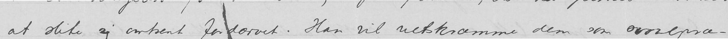at slite sig omtrent fordærvet. Han vil vetskræmme dem som svovelpræ-
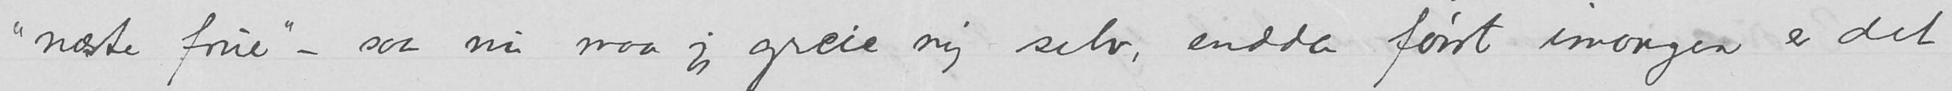«næste frue» – saa nu maa jeg greie mig selv, endda først imorgen er det
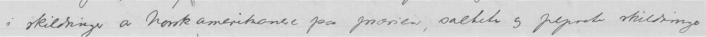i skildringer av Nordamerikanere paa prærien, saltete og peprete skildringer
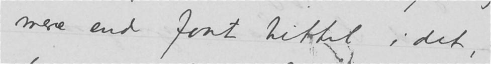mere end faat tittet i det,
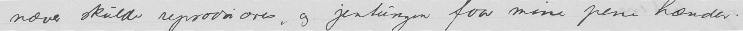næver skulde reproduseres, og jentungen faar mine pene hænder.
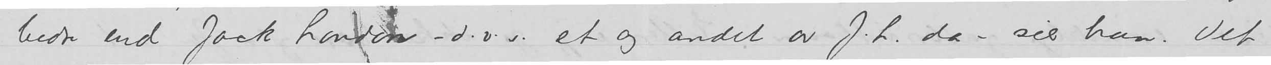bedre end Jack London d.v.s. et og andet av J.L. da – sier han. Det
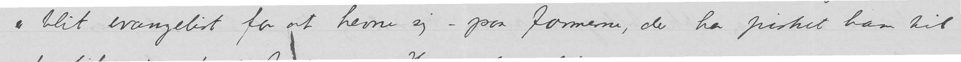er blit evangelist for at hevne sig – paa farmerne, der har pisket ham til
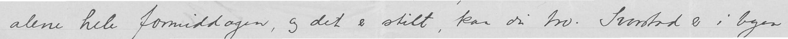alene hele formiddagen, og det er stilt, kan du tro. Svarstad er i byen
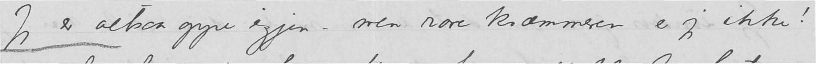Jeg er altsaa oppe igjen – men rare kræmmeren er jeg ikke!
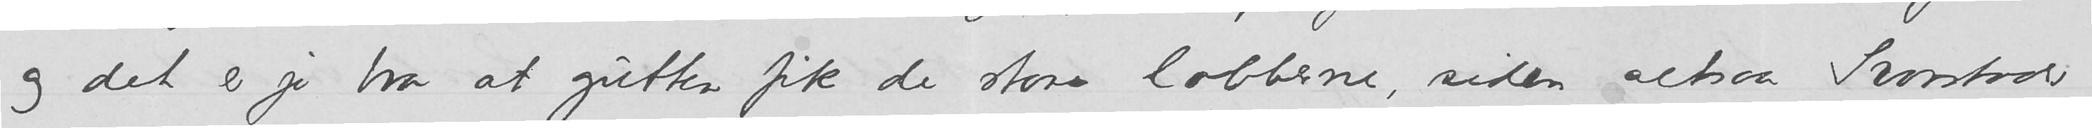og det er jo bra at gutten fik de store labberne, siden altsaa Svartstads
